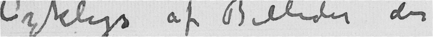Cyklys af Billeder der
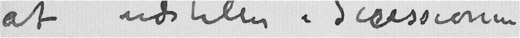at udstille i Secessionen
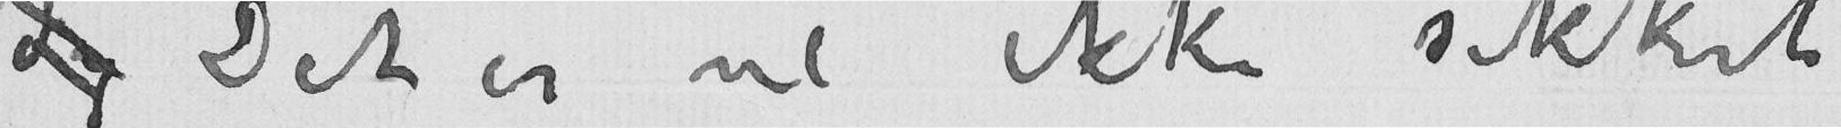Jeg Det er vel ikke sikkert
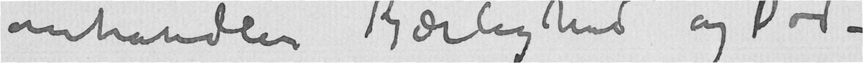omhandler Kjærlighed og Død –
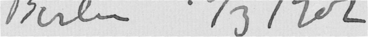Berlin 18/3 1902
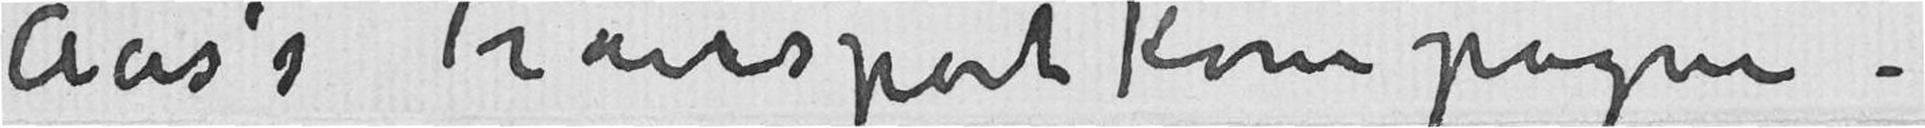Aas’s Transportkompagni.
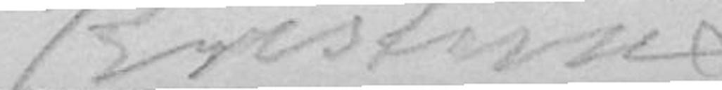Kristine
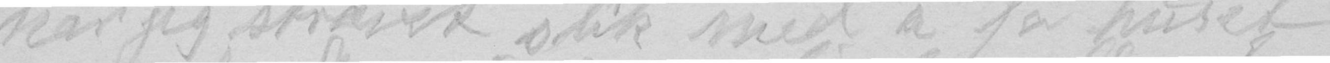har jeg strævet slik med å få huset
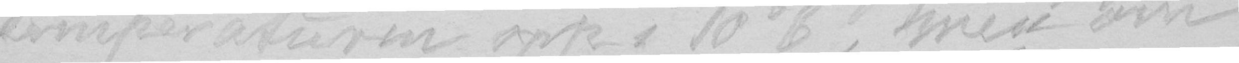temperaturen opp i 10 ⁰ C, men om
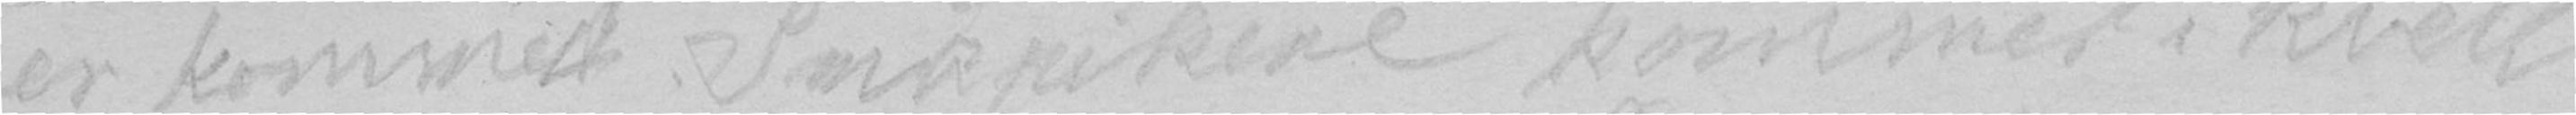er kommet. Småpikene kommer i kveld
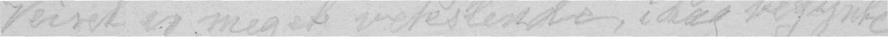Veiret er meget vekslende, i dag begynte
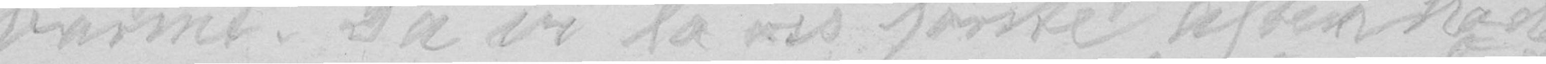varmt. Da vi la oss første aften hadde
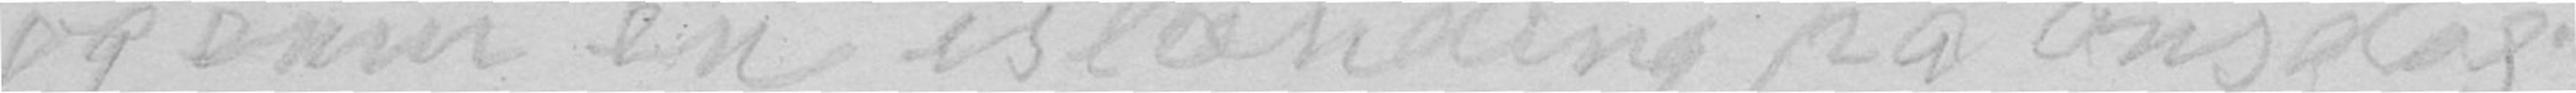og ennu en islænding på onsdag.
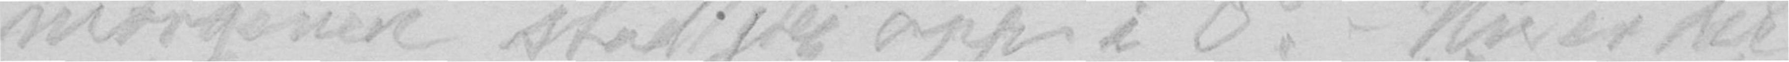morgenen stod jeg opp i 0 ⁰. - Nu er det
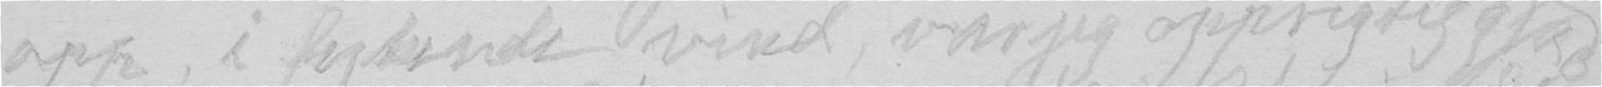opp, i fykende vind, var jeg opprigtig glad
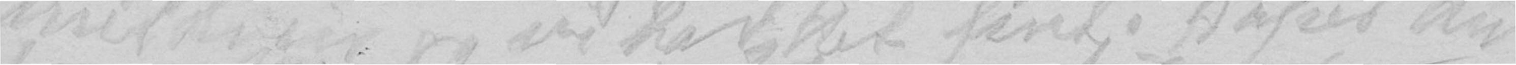mildveir og vi har det fint. Håper du
